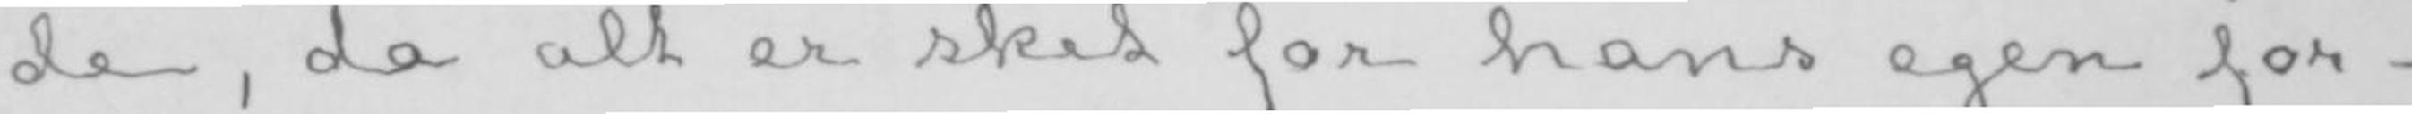de, da alt er sket for hans egen for-
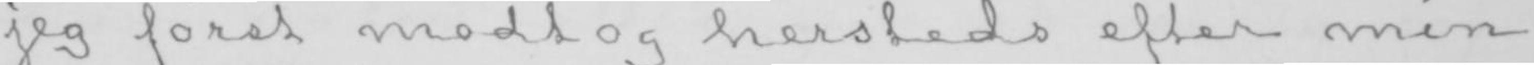jeg først modtog hersteds efter min
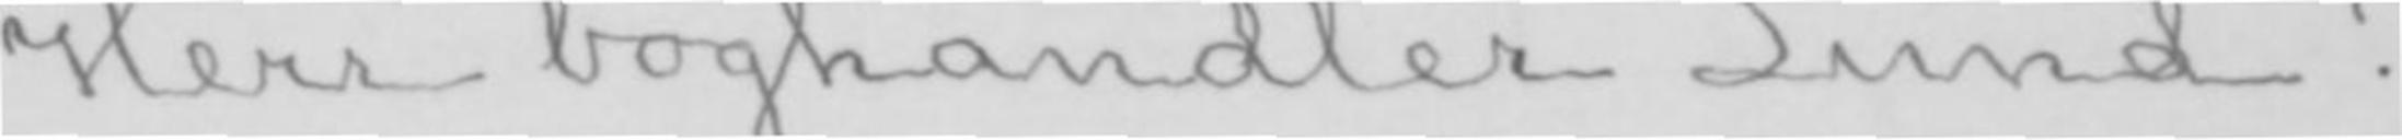Herr boghandler Lund!
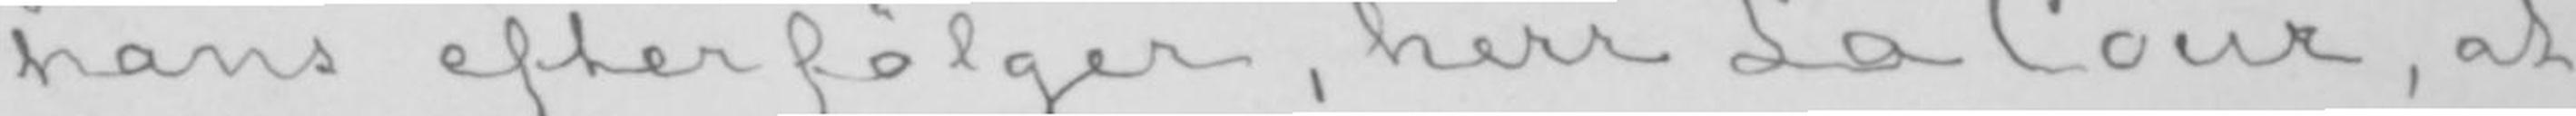hans efterfølger, herr La Cour, at
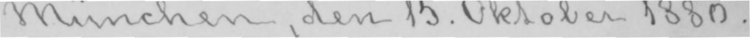München, den 15. Oktober 1880.
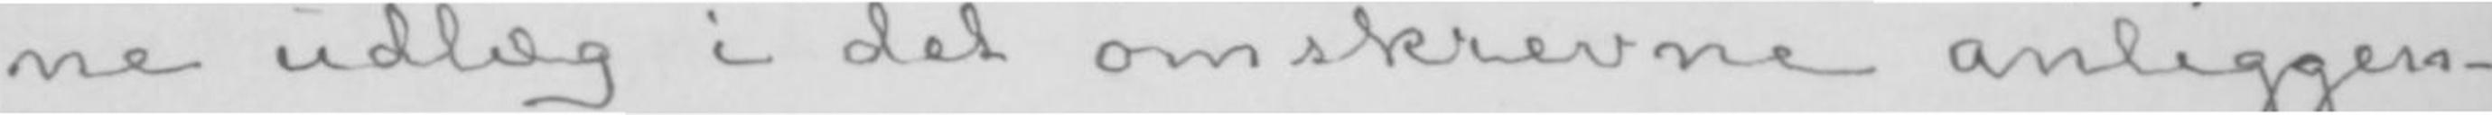ne udlæg i det omskrevne anliggen-
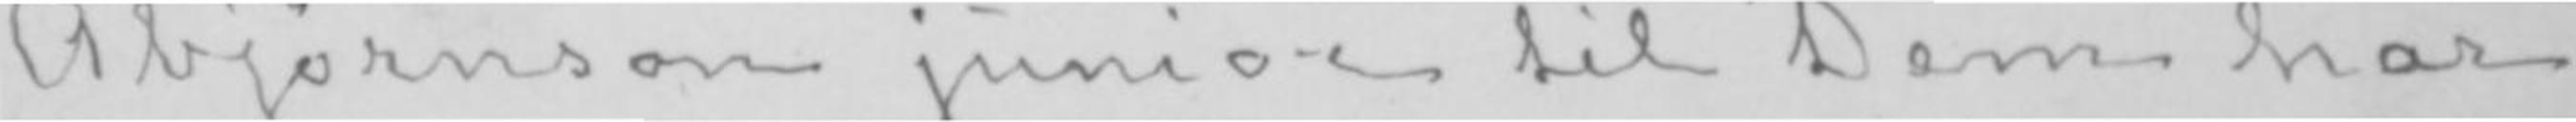Åbjörnson junior til Dem har
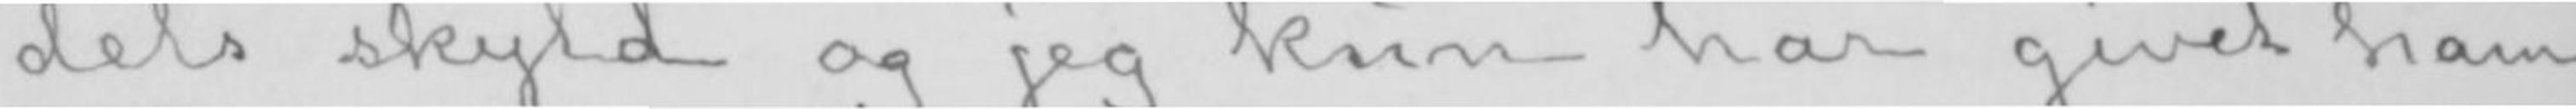dels skyld og jeg kun har givet ham
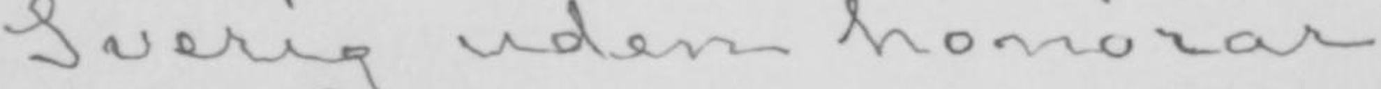Sverig uden honorar.
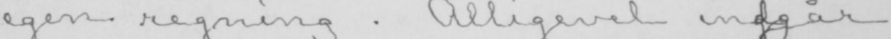egen regning. Alligevel indgår
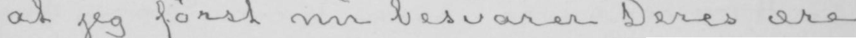at jeg først nu besvarer Deres ære-
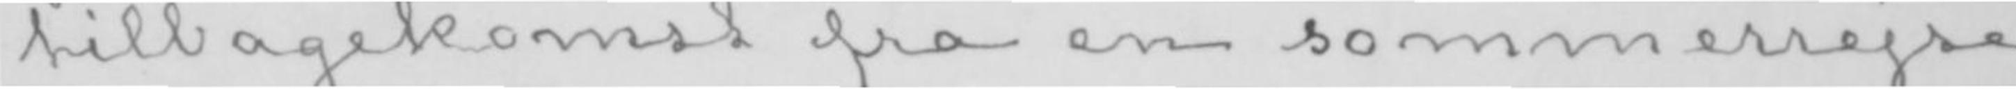tilbagekomst fra en sommerrejse
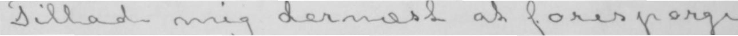Tillad mig dernæst at forespørge
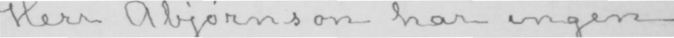Herr Åbjörnson har ingen
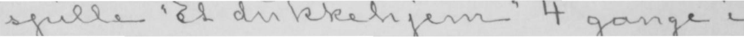spille «Et dukkehjem» 4 gange i
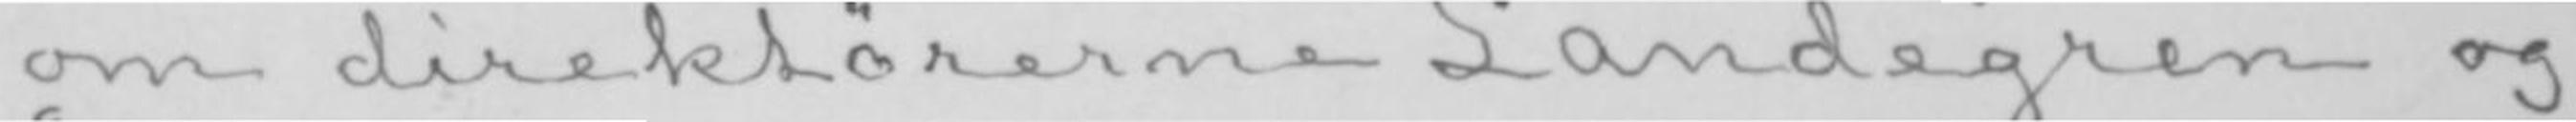om direktørerne Landegren og
